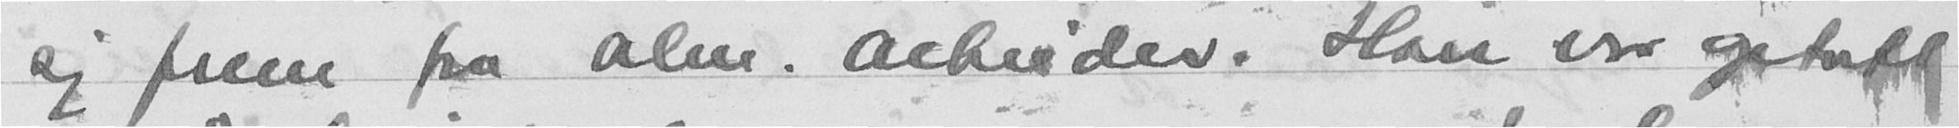sig frem fra alen. Arbeider. Han var optatt
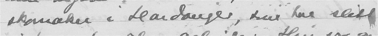skomaker i Hardanger, som har slitt
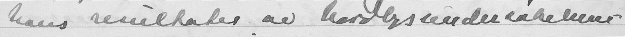hans resultater av nordlysundersøkelsene.
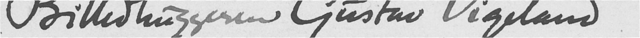Billedhuggeren Gustav Vigeland
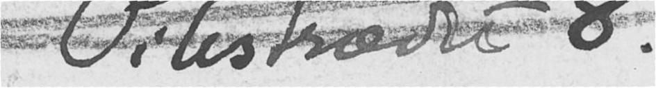Pilestrædet 8.
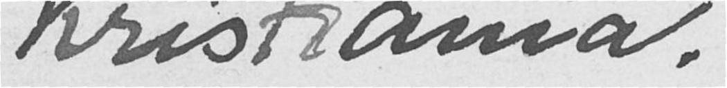Kristiania.
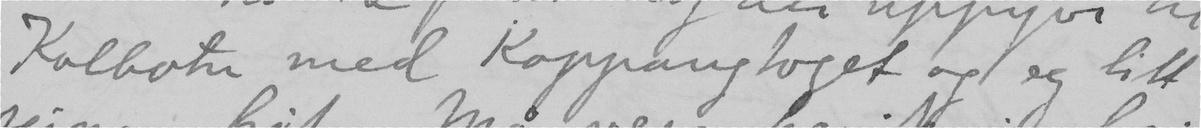Kolbotn med Koppangtoget og eg litt
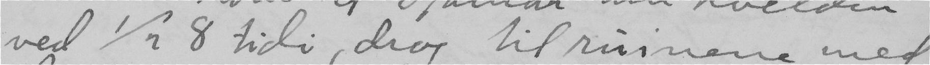ved ½ 8 tidi, drog til ruinene med
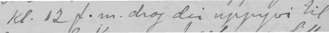Kl. 12 f.m. drog dei uppyvi til
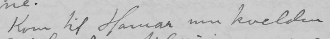Kom til Hamar um kvelden
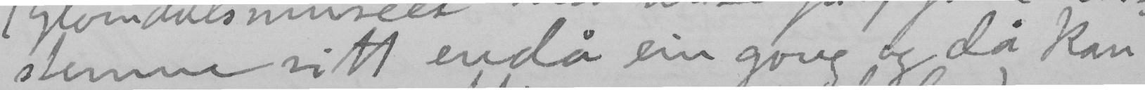stemne sitt endå ein gong og då kan
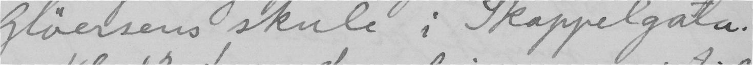Glöersens skule i Skappelgata.
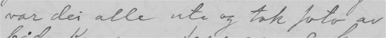var dei alle ute og tok foto av
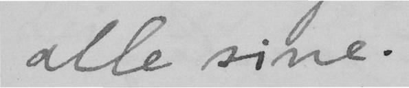alle sine.
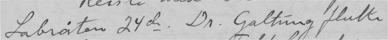Labråten 24de. Dr. Galtung flutte
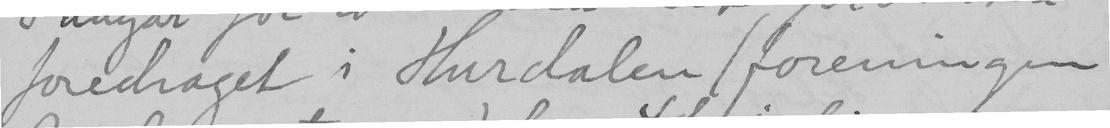foredraget i Hurdalen (foreningen
